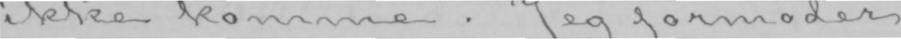ikke komme. Jeg formoder
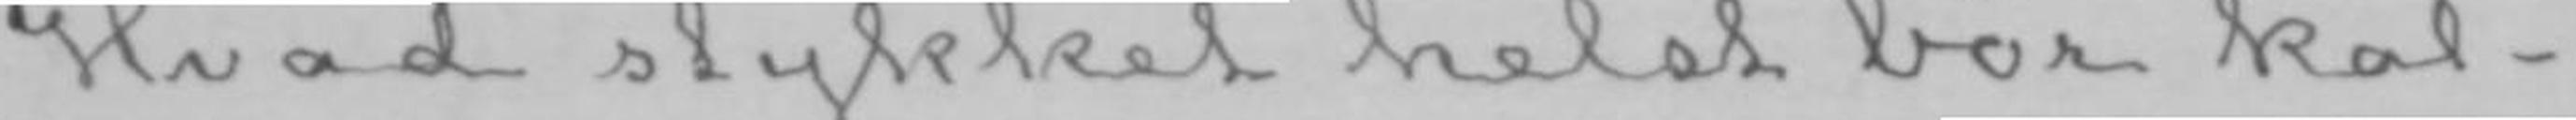Hvad stykket helst bør kal-
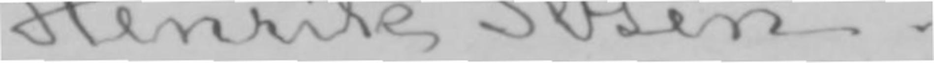Henrik Ibsen.
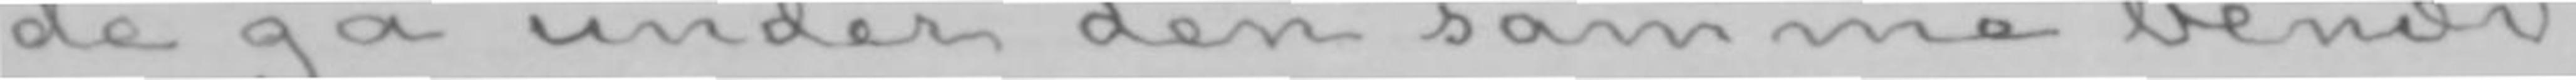de gå under den samme benæv-
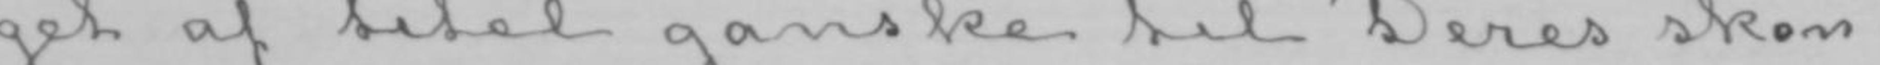get af titel ganske til Deres skøn.
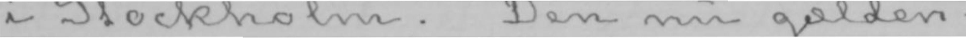i Stockholm. Den nu gælden-
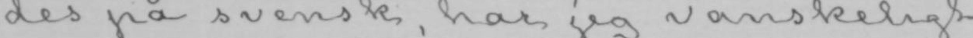des på svensk, har jeg vanskeligt
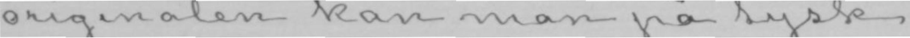originalen kan man på tysk
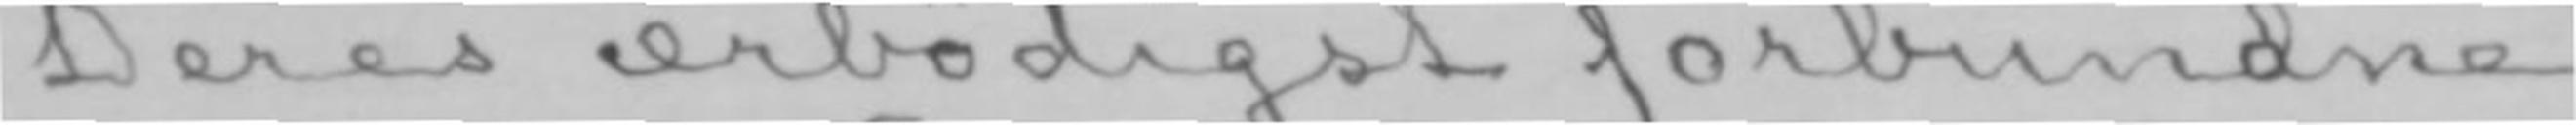Deres ærbødigst forbundne
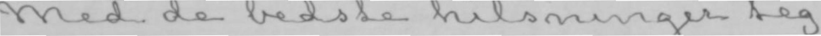Med de bedste hilsninger teg-
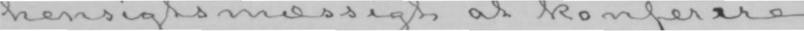hensigstsmæssigt at konferere
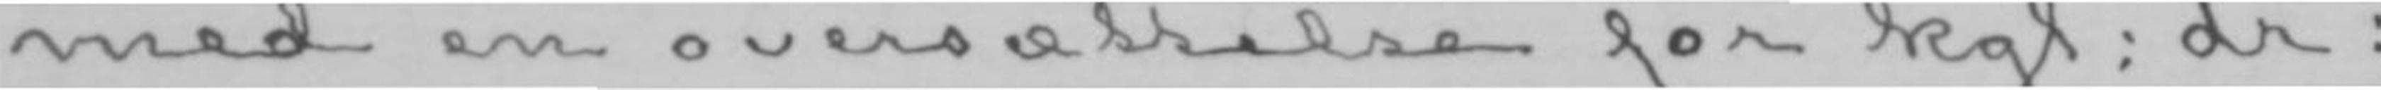med en oversættelse for kgl: dr:
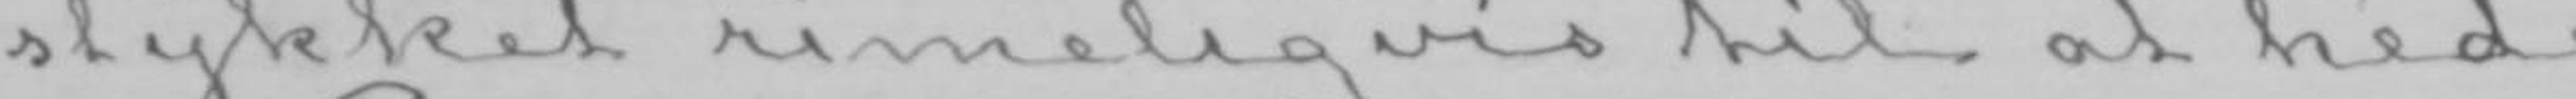stykket rimeligvis til at hede
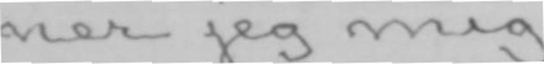ner jeg mig
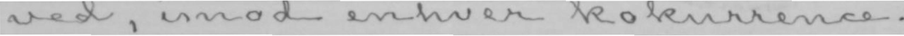ved, imod enhver kokurrence.
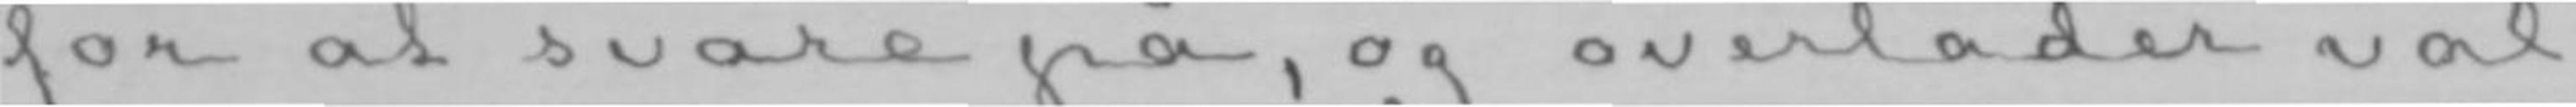for at svare på, og overlader val-
